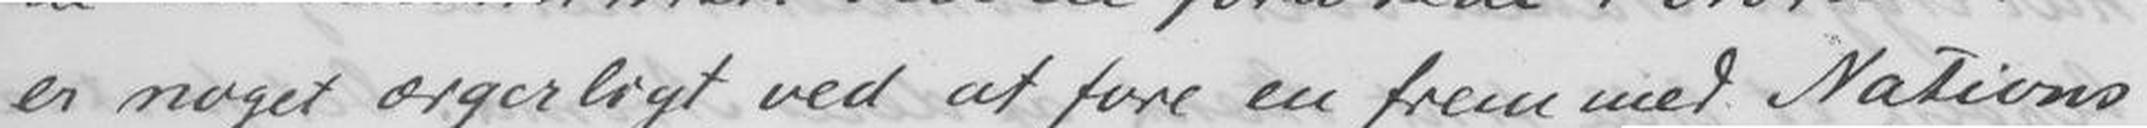er noget ærgerligt ved at fore en fremmed Nations
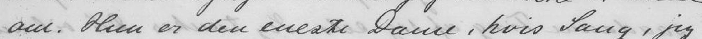om. Hun er den eneste Dame, hvis Sang, jeg
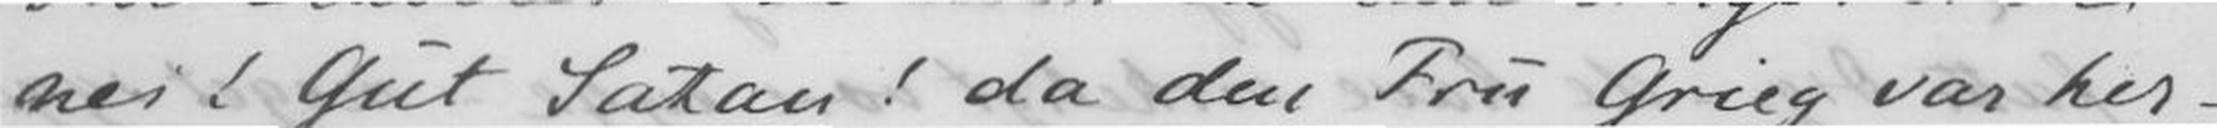nei! Gut Satan! da den Fru Grieg var her -
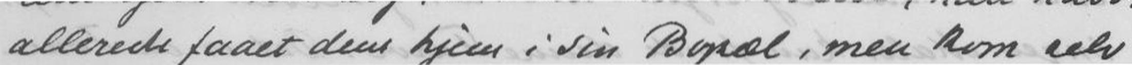allerede faaet dem hjem til sin Bopel, men kom selv
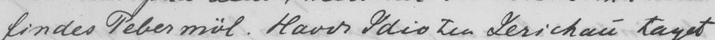findes Pebermøl. Havde Idioten Jerichau taget
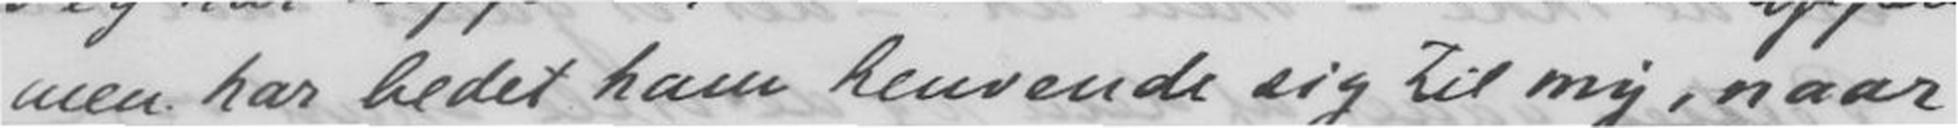men har bedet ham henvende sig til mig, naar
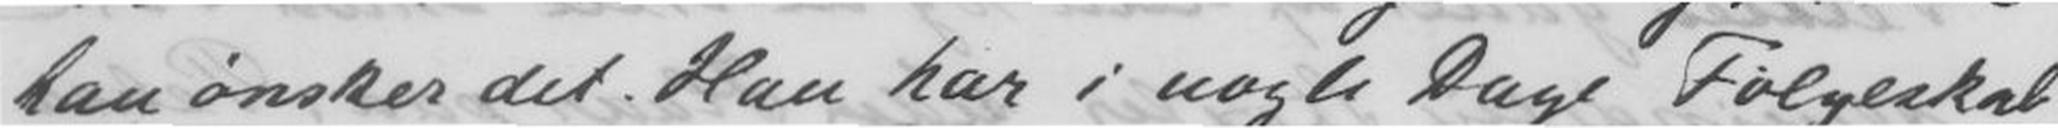han ønsker det. Han har i nogle Dage Følgeskab
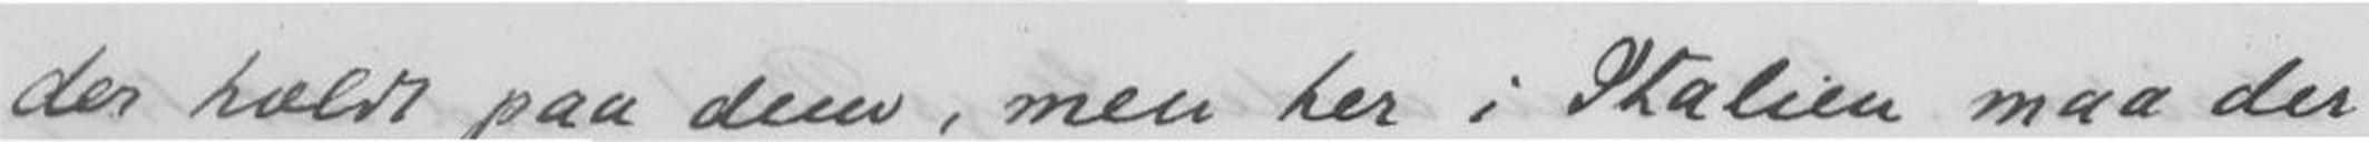der holdt paa dem, men her i Italien maa der
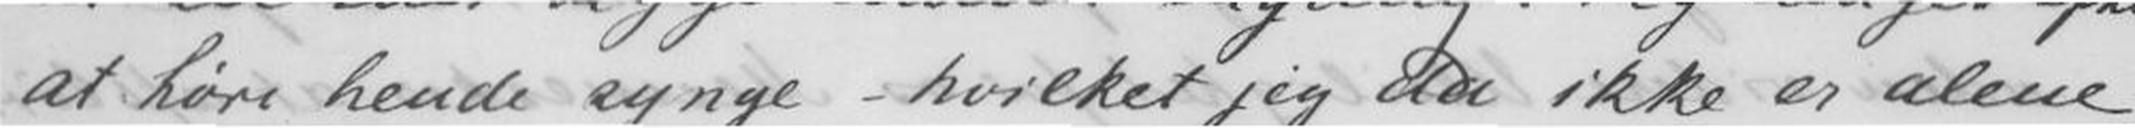at høre hande synge - hvilket jeg da ikke er alene
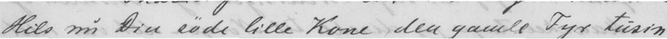Hils nu Din søde lille Kone den gamle Fyr tus-
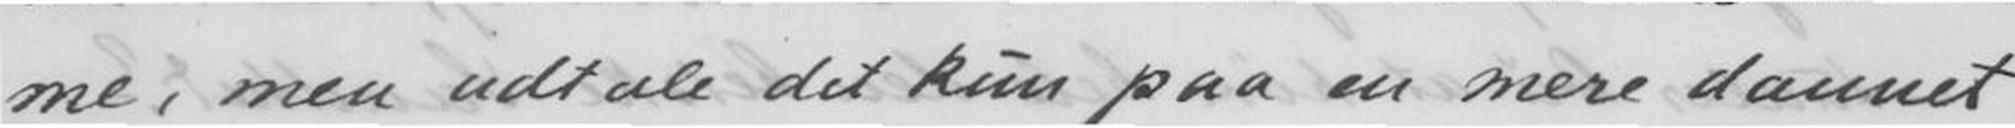me, men udtale det kun paa en mere dannet
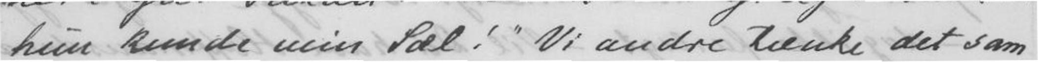hun kunde min Sæl! Vi andre tænke det sam-
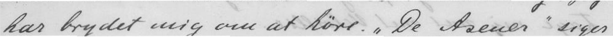har brydet mig om at høre. "De Asener" siger
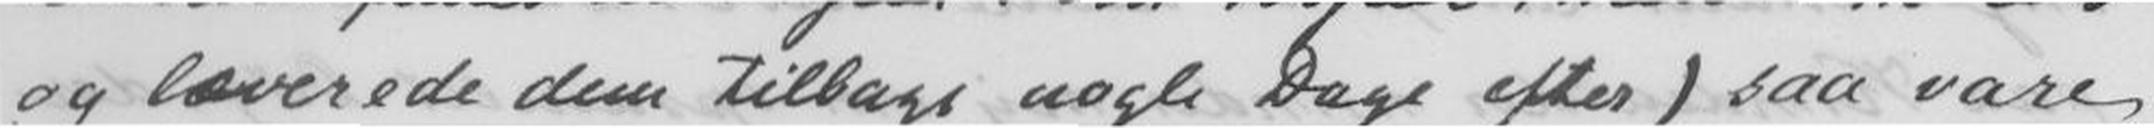og læverede dem tilbage nogle Dage efter) saa var
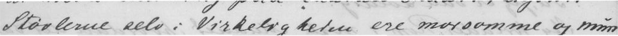Støvlerne selv i Virkligheden ere morsomme og mun-
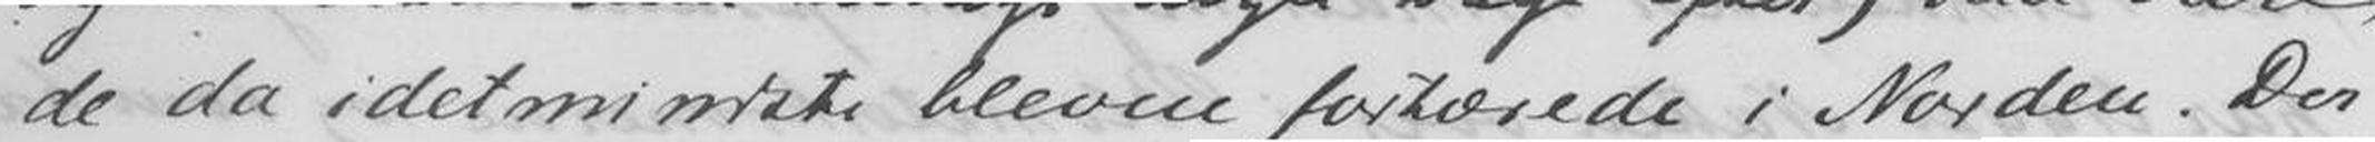de da idetminsdte bleven fortærede i Norden. Der
